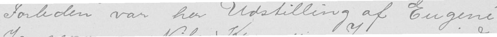Forleden var her Udstilling af Eugene
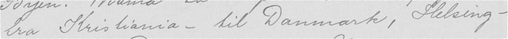fra Kristiania - til Danmark, Helsing-
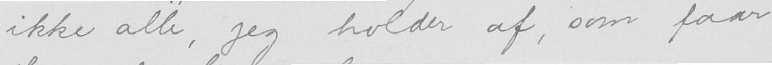ikke alle, jeg holder af, som faar
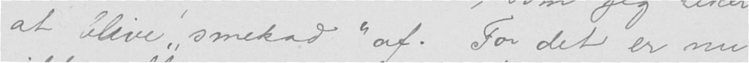at blive "smekad" af. For det er nu
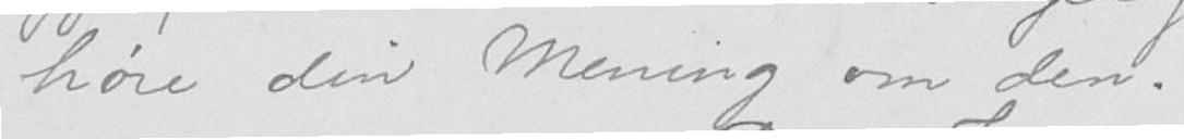høre din Mening om den.
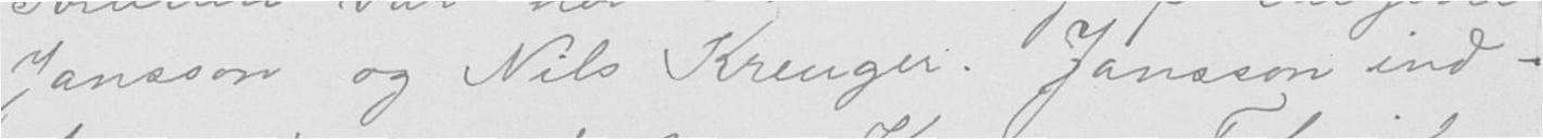Jansson og Nils Kreuger. Jansson ind-
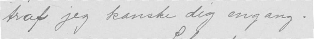traf jeg kanske dig engang.
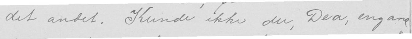det andet. Kunde ikke du, Dea, engang
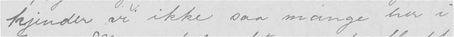kjender vi ikke saa mange her i
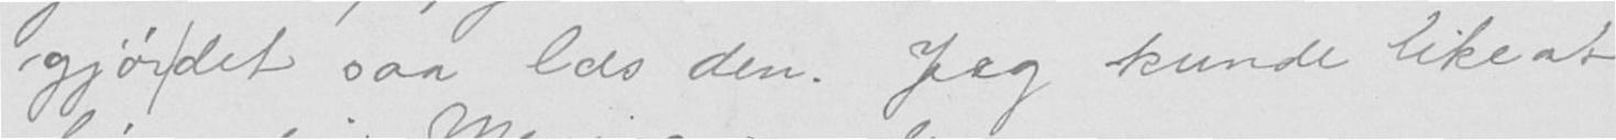gjør det saa læs den. Jeg kunde like at
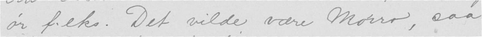ør f.eks. Det vilde være Morro, saa
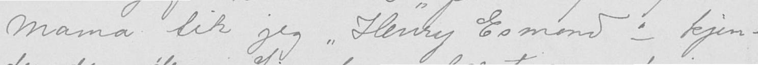Mama fik jeg "Henry Esmond" - kjen-
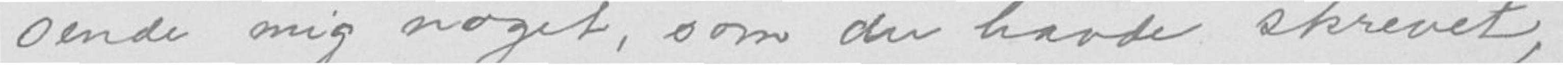sende mig noget, som du havde skrevet,
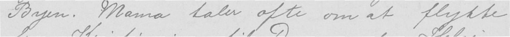Byen. Mama taler ofte om at flytte
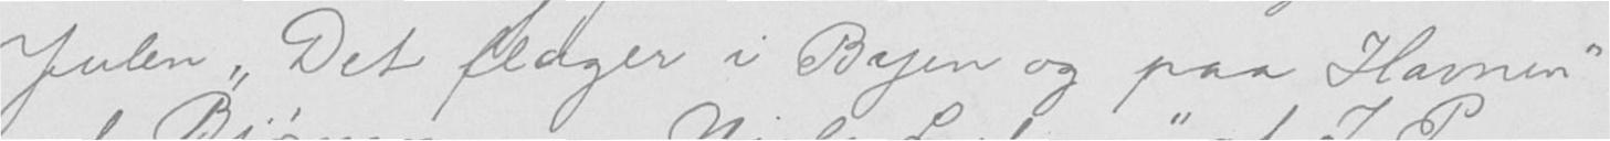Julen. "Det flager i Byen og paa Havnen"
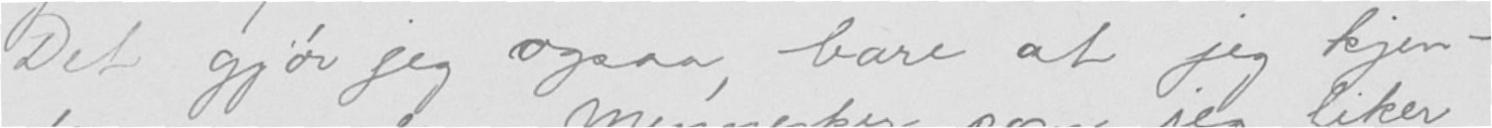Det gjør jeg ogsaa, bare at jeg kjen-
